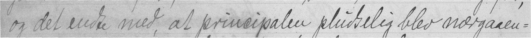og det endte med, at principalen pludselig blev nærgaaen-
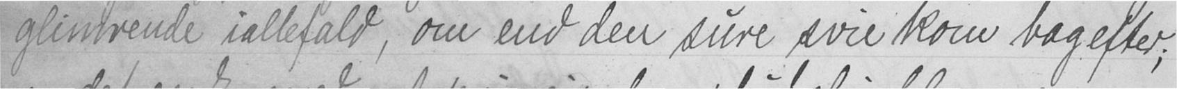glimrende iallefald, om end den sure svie kom bagefter;
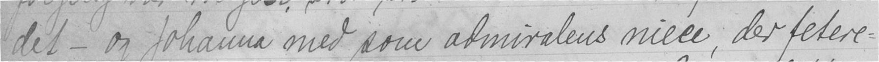det - og Johanna med som admiralens niece, der fetere-
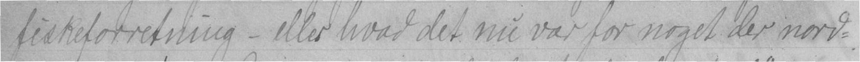fiskeforretning - eller hvad det nu var for noget der nord-
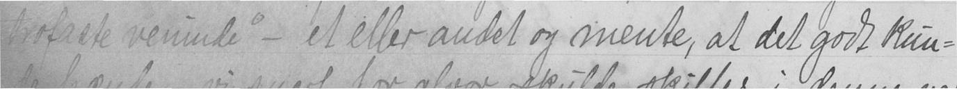trofaste veninde" - et eller andet og mente, at det godt kun-
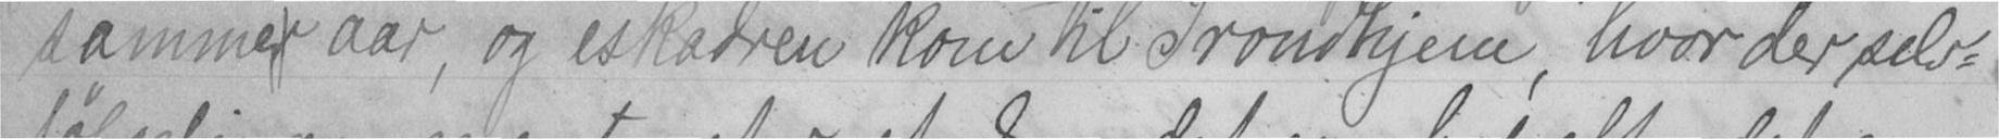sammer aar, og eskadren kom til Trondhjem, hvor der selv-
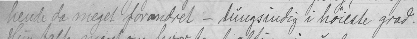hende da meget forandret - tungsindig i høieste grad.
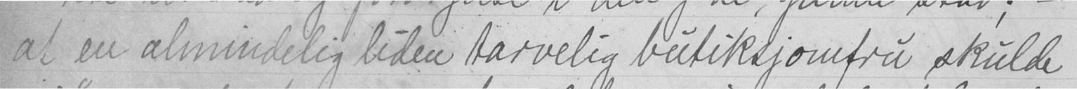at en almindeng liden tarvelig butiksjomfru skulde
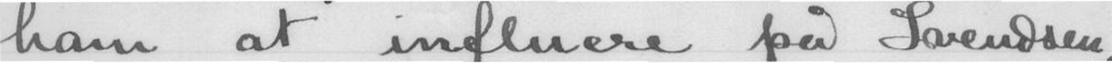ham at influere på Svendsen,
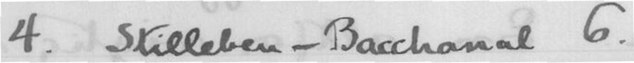4. Stilleben-Bacchanal 6.
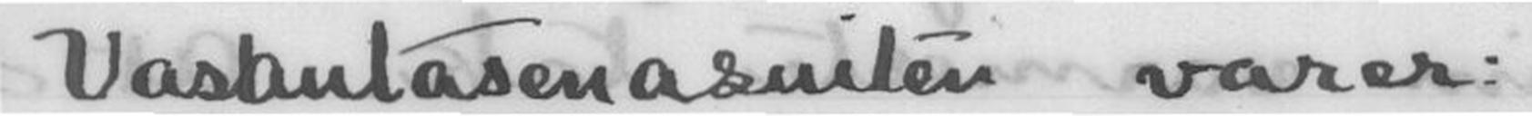Vasantasenasuiten varer:
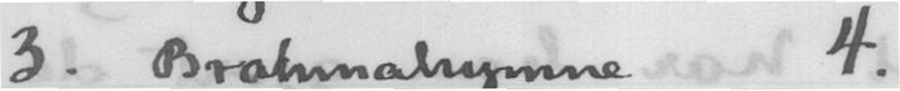3. Brahmahymne 4.
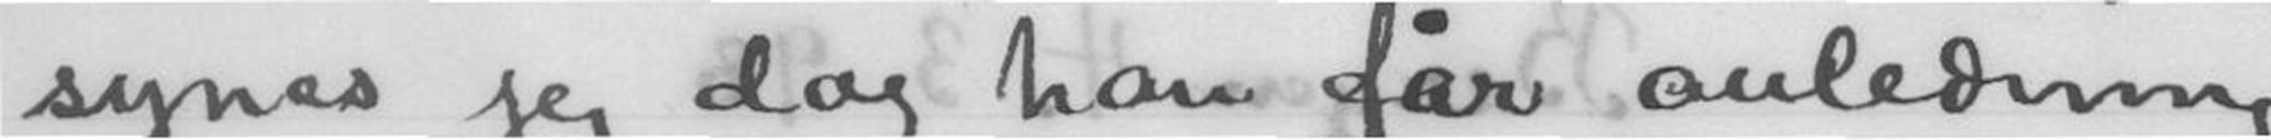synes jeg dog han får anledning
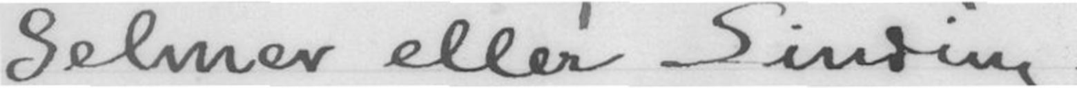Selmer eller Sinding.
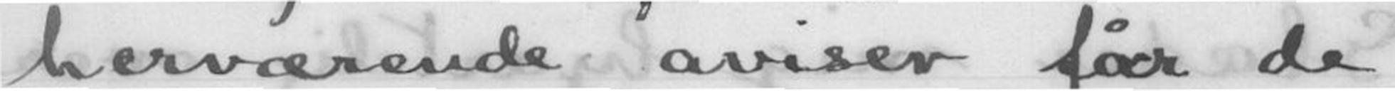herværende aviser får de
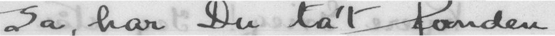Ja, har Du tatt fanden
